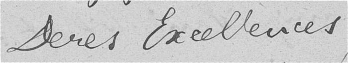Deres Excellences
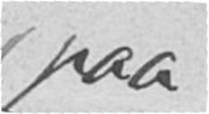paa
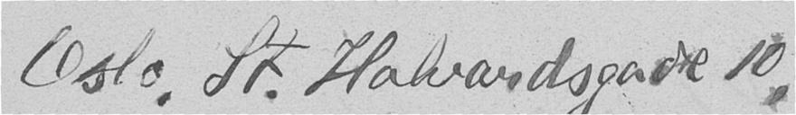Oslo, St. Halvardsgade 10,
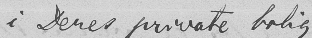i Deres private bolig
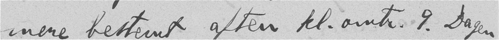mere bestemt aften kl. omtr. 9. Dagen
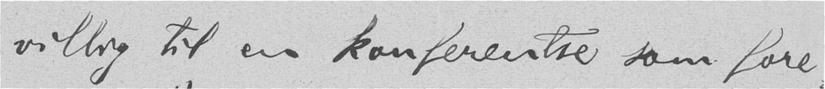villig til en konferentse som fore-
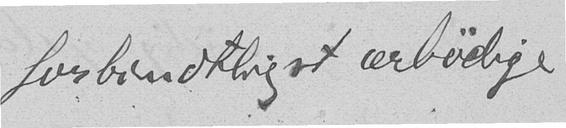forbindtligst ærbødige
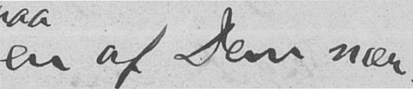en af Dem nær-
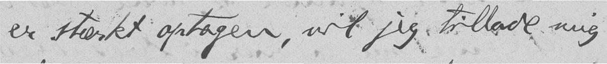er stærkt optagen, vil jeg tillade mig
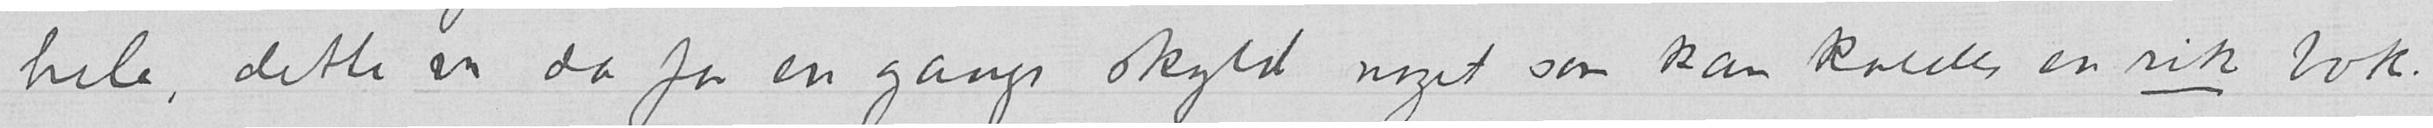hele, dette var da for en gangs skyld noget som kan kallles en rik bok.
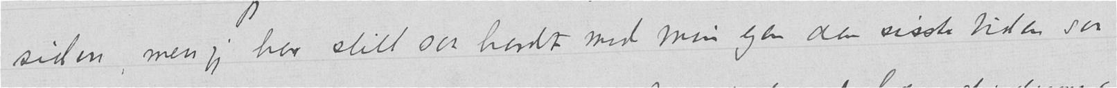siden, men jeg har slitt saa hardt med min egen den sidste tiden saa
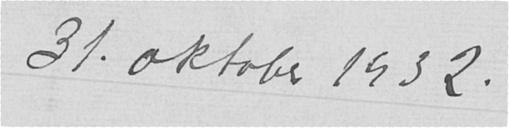31. oktober 1932.
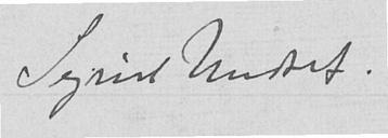Sigrid Undset.
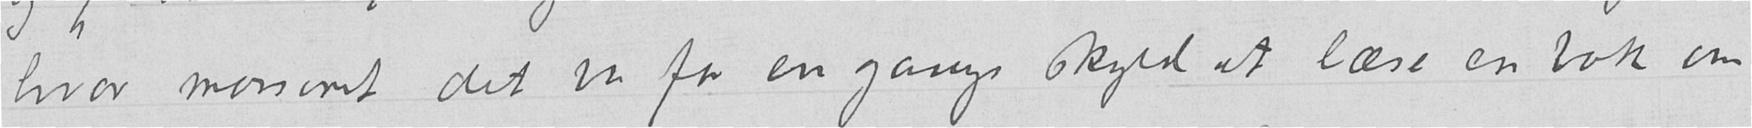hvor morsomt det var for en gangs skyld at læse en bok om
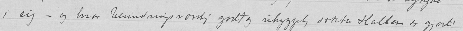i sig – og hvor beundringsværdig godt og uhyggelig doktor Hallem er gjort!
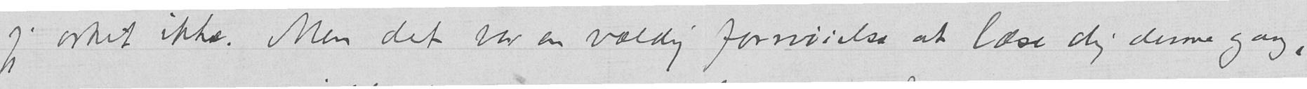jeg orket ikke. Men det var en vældig fornøielse at læse dig denne gang,
